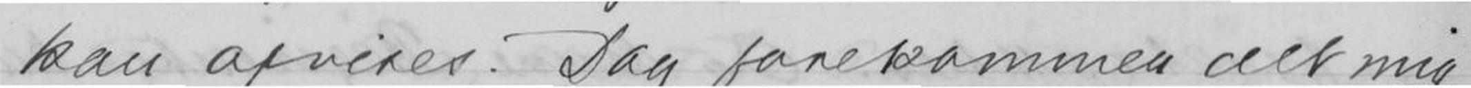kan afvikes. Dag forekommer det mig
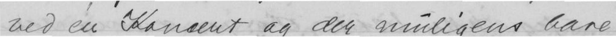ved en Konsert og det muligens bare
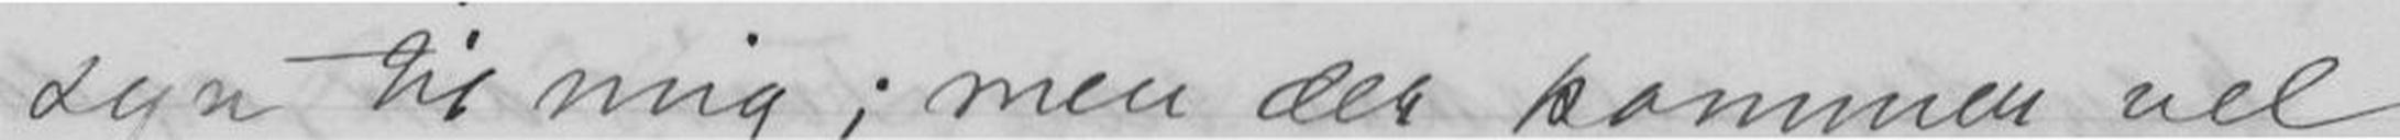syn til mig; men det kommer vel
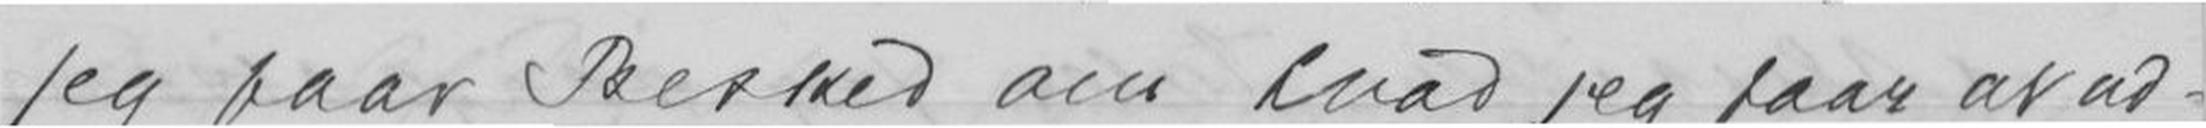jeg faar Besked om hvad jeg faar at ud-
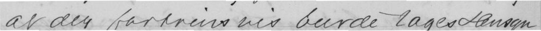at det fortrinsvis burde tages hensyn
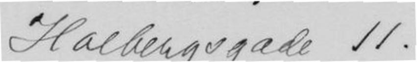Holbergsgade 11
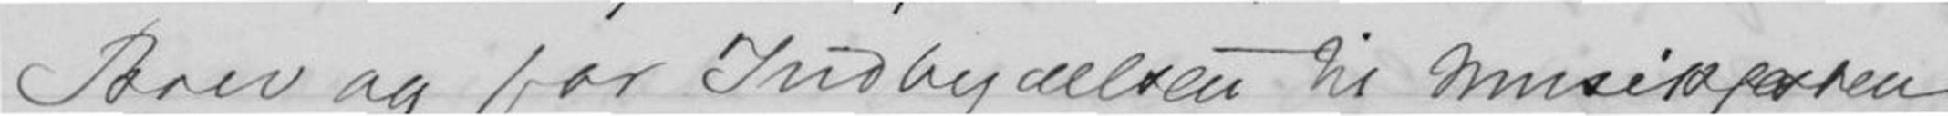Brev og for Indbydelsen til musik
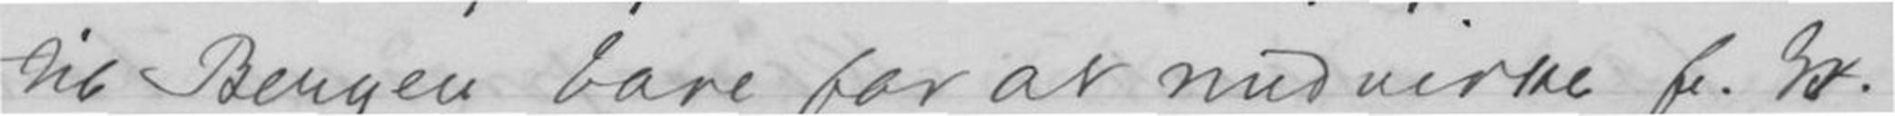til Bergen bare for at medvirke f.Ex.
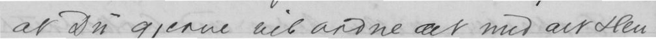at Du gjerne vil ordne det med av Hen
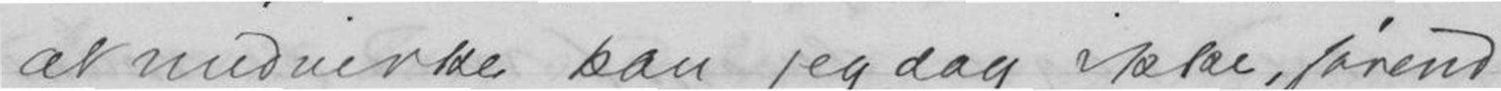at medvirke kan jeg dog ikke,
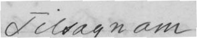Tilsagn om
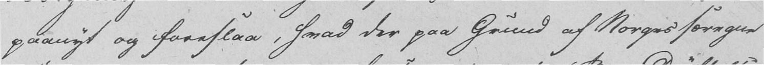paanyt og foreslaa, hvad der paa Grund af Norges særegne
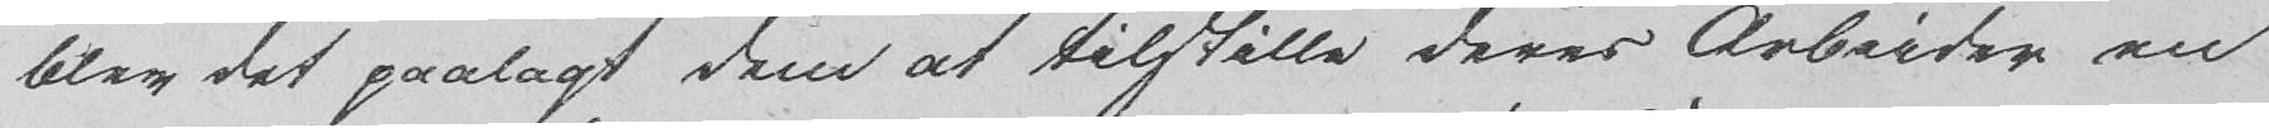blev det paalagt dem at tilstille deres Arbeider en
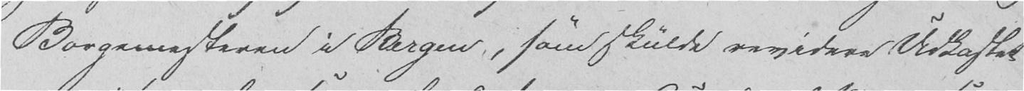Borgermesteren i Bergen, som skulde revidere Udkastet
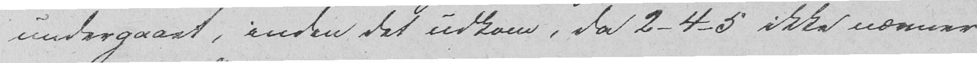undergaaet, inden det udkom, da 2-4-5 ikke nævner
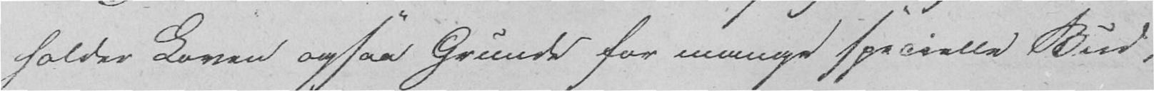holder Loven ogsaa Grunde for mange specielle Bud,
